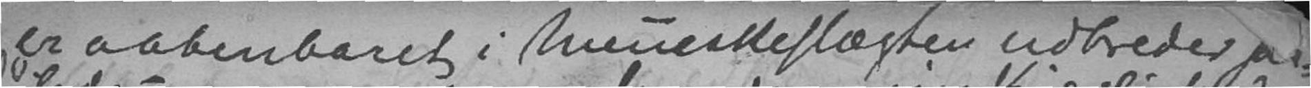er aabenbaret i Menneskeslægten udbreder saa-
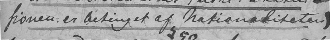sionen er betinget af Nationaliteten)
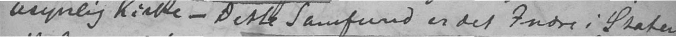usynlig Kilde – Dette Samfund er det Indre i Staten
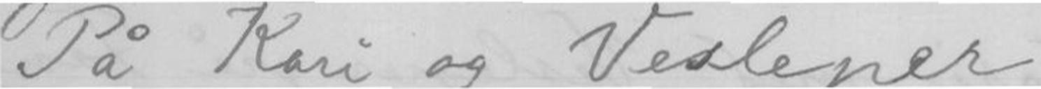På Kari og Vesleper
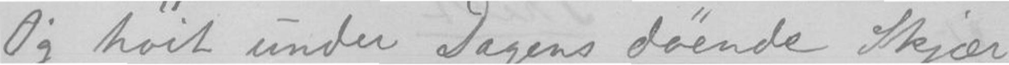Og høit under Dagens døende skjær
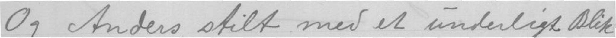Og Anders stilt med et undeligt Blik
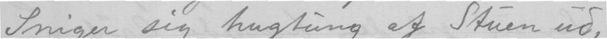Smiger sig hugtung af Stuen ud,
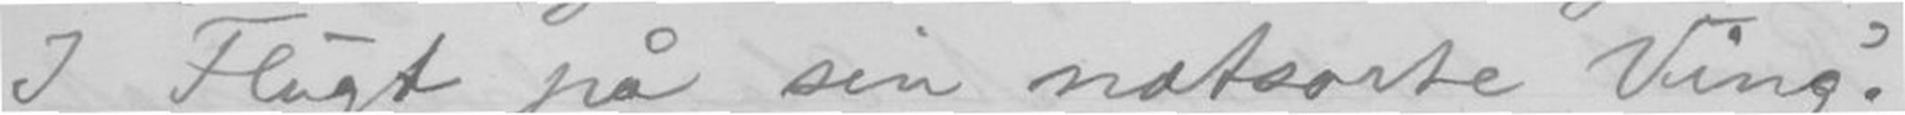I Flugt på sin natsorte Ving.
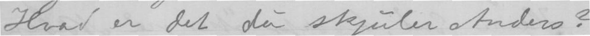Hvad er det du skjuler Anders?
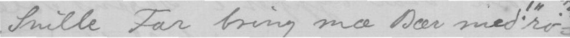- "Snille Far bring mæ Bær med!" ro-
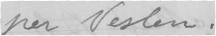er Veslen.
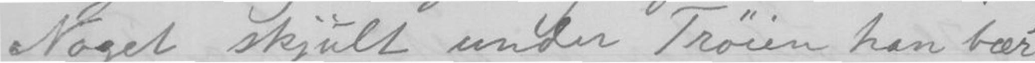Noget skjult under Trøien han bær
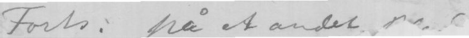Forts. på et andet
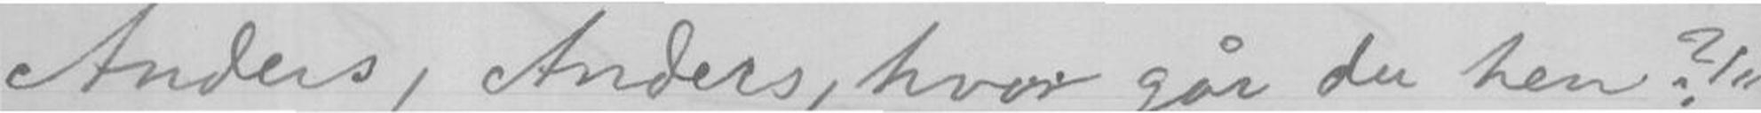"Anders, Anders, hvor går du hen?!"
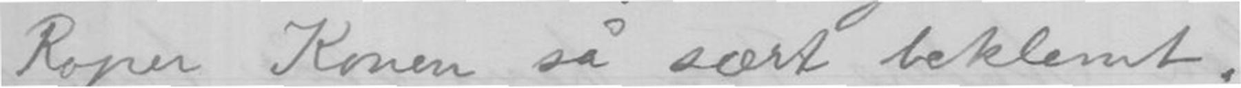Roper Konen så sært beklemt.
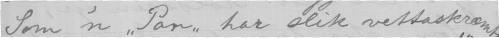Som 'n "Par" har slik vettaskræmt!
